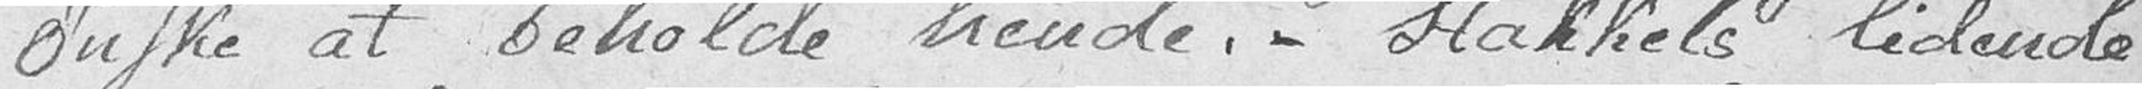Ønske at beholde hende. – Stakkels lidende
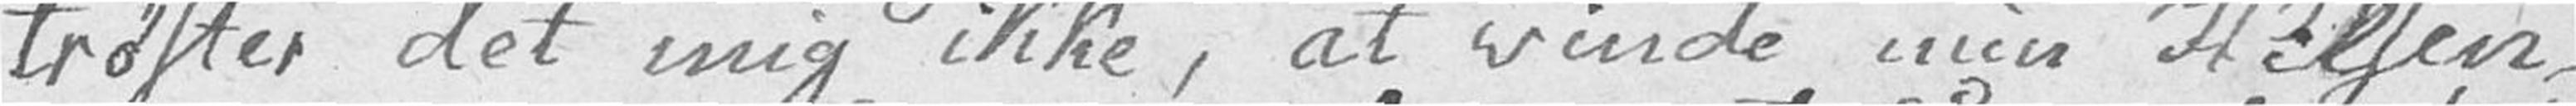trøster det mig ikke, at vinde min Hilsen
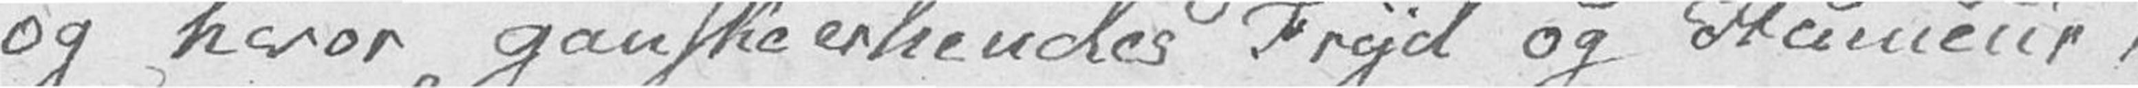og hvor ganske er hendes Fryd og Humeur,
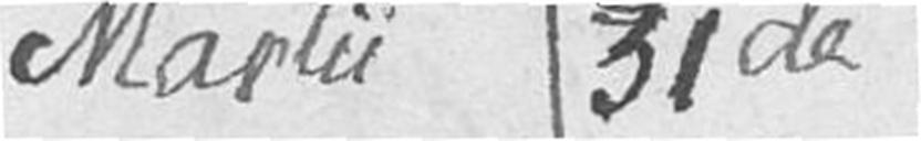Martii 31de
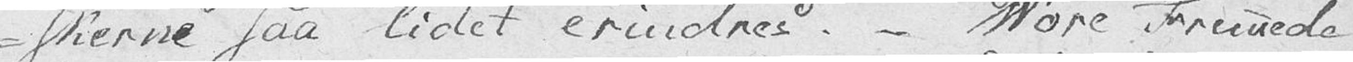-skerne saa lidet erindres. – Vore Fremmede
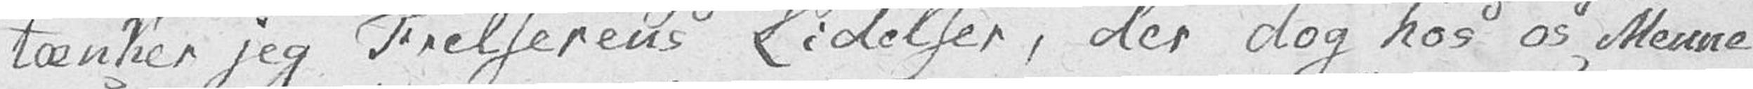tænker jeg Frelserens Lidelser, der dog hos os Menne
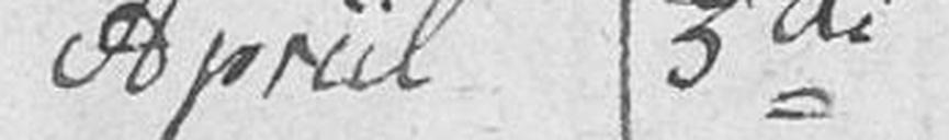Apriil 3di
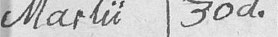Martii 30d.
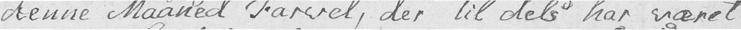denne Maaned Farvel, der til dels har været
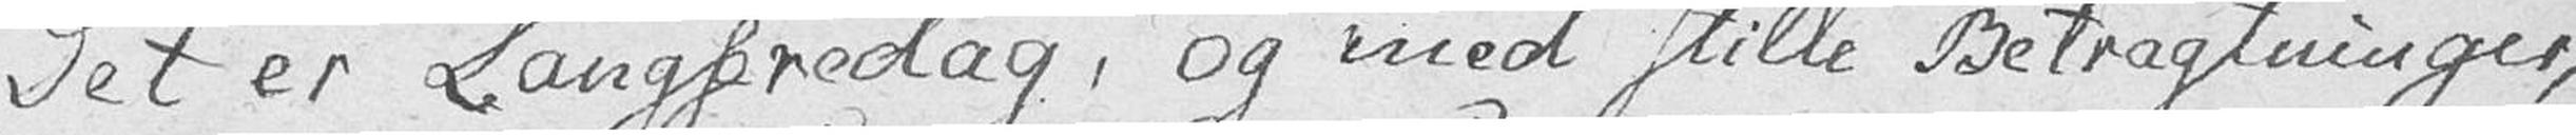Det er Langfredag, og med stille Betragtninger,
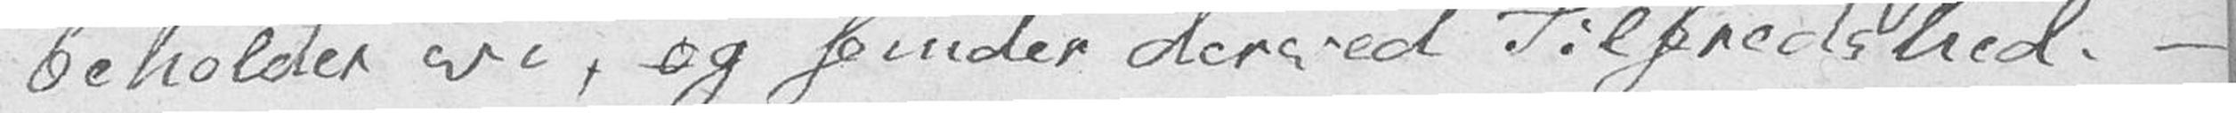beholder vi, og finder derved Tilfredshed. –
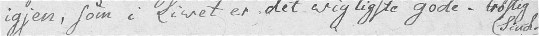igjen, som i Livet er det vigtige gode. trøstig Sind.
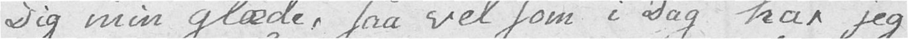Dig min glæde, saa vel som i Dag har jeg
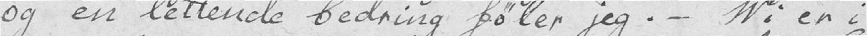og en lettende bedring føler jeg. – Vi er i
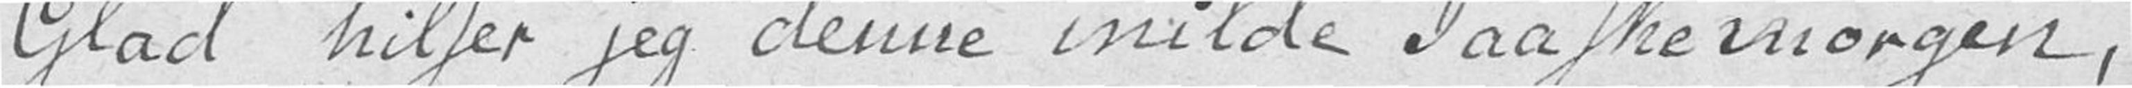Glad hilser jeg denne milde Paaskemorgen,
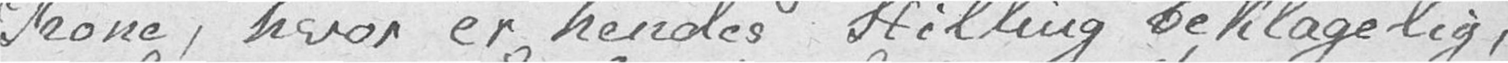Kone, hvor er hendes Stilling beklagelig,
